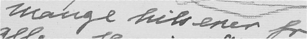Mange hilsener fra os
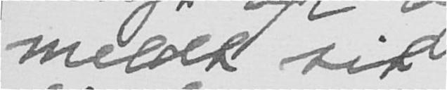meldt sit
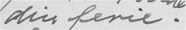din ferie.
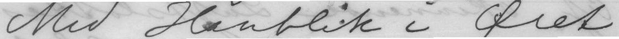Med Hånblik i Øiet
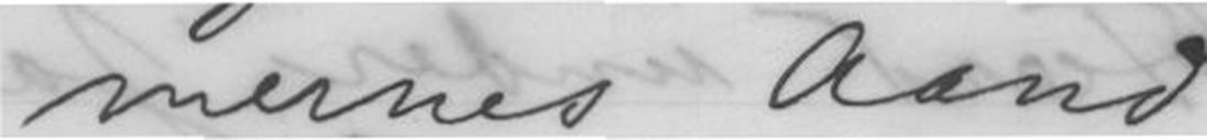mernes Aand
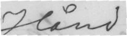Hånd
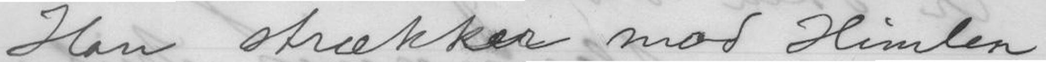Han strækker mod Himlen
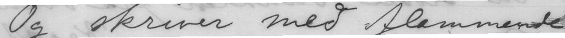Og skriver med flammende
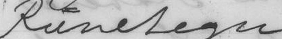Runetegn
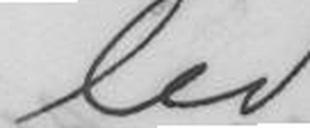led
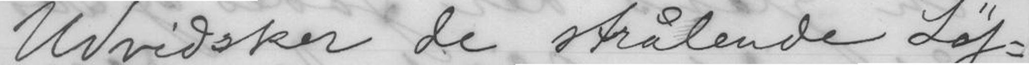Udvidsker de strålende Løf-
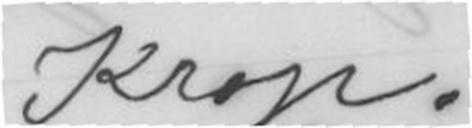Krop.
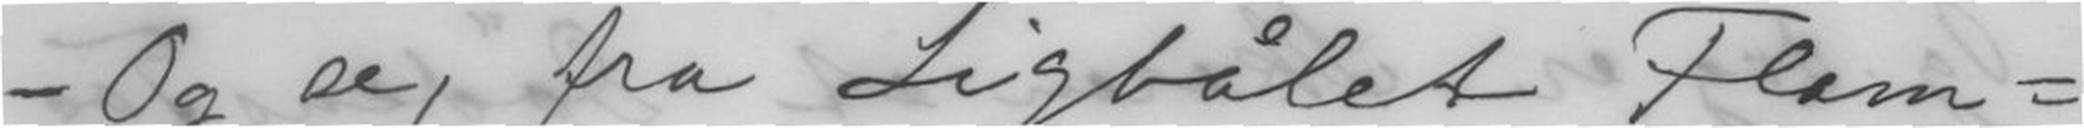- Og se, fra Ligbålet Flam-
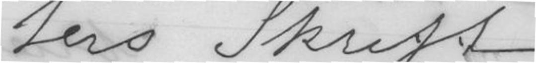ers Skrift
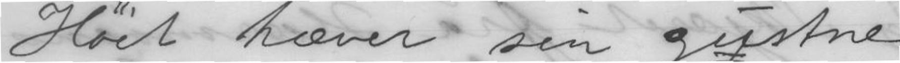Høit hæver sin gustne
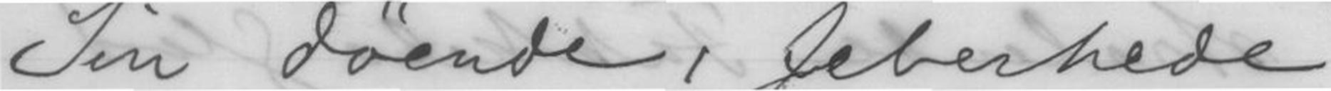Sin døende, feberhede
